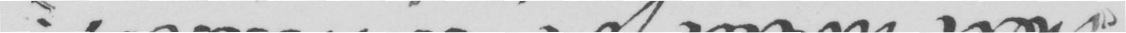Men hvad for en smuds-
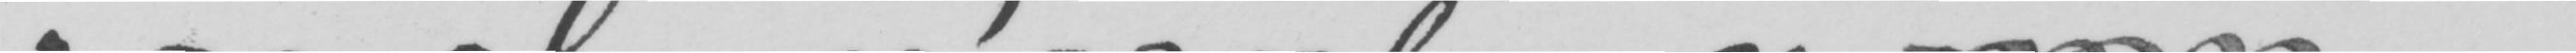- men alligevel, alligevel!
